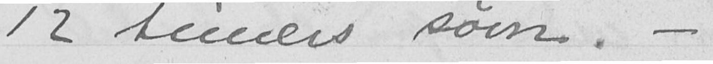12 timers søvn. -
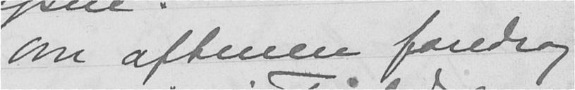Om aftenen foredrag
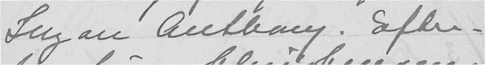Suzan Anthony. Efter
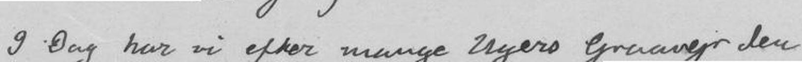I Dag har vi efter mange uger Graavejr den
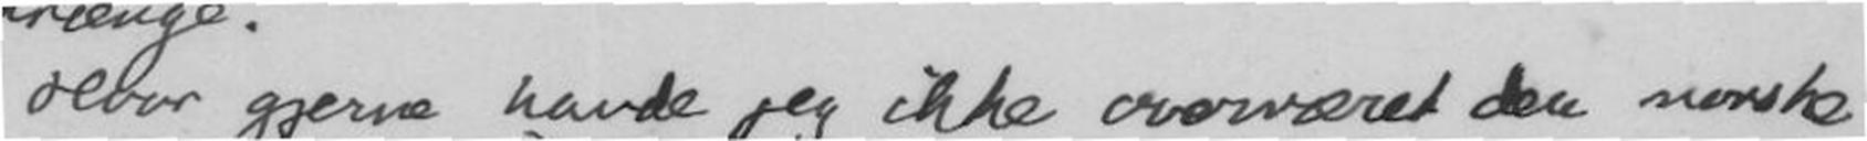Hvor gjerne havde jeg ikke overværet den norske
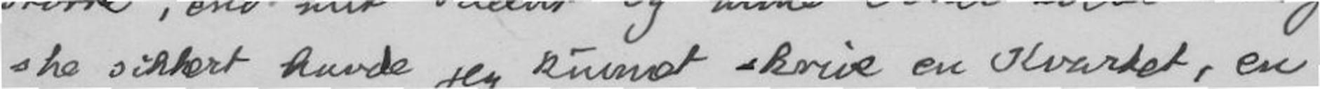ske sikkert havde jeg kunnet skrive en Kvartet, en
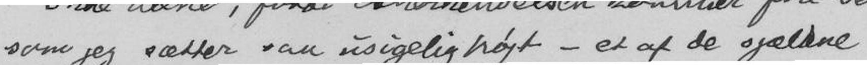som jeg sætter saa usigelig højt - et af de sjældne
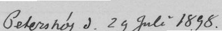Petershøj 29 Juli 1898
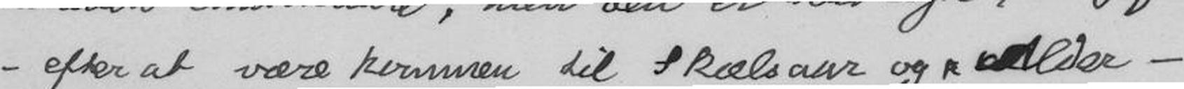- efter at være kommen til Skælsaar og alder -
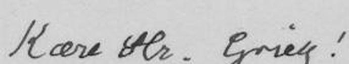Kære Hr. Grieg!
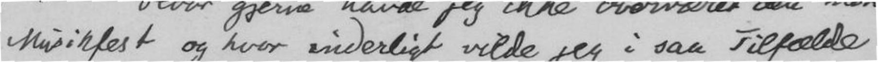Musikfest og hvor inderligt vilde jeg i saa Tilfælde
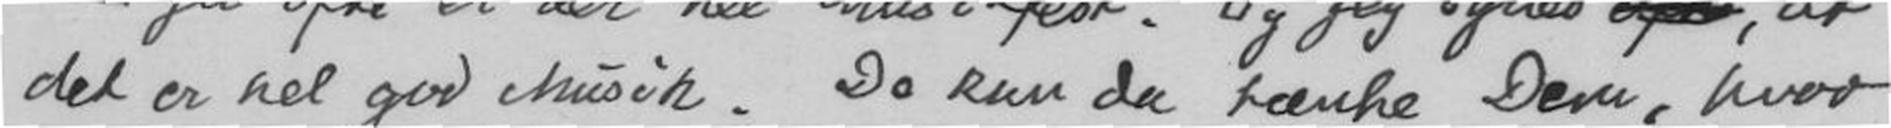det er hel god musik. Da kan da tænke Dem, hvor
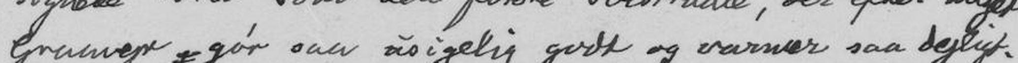Graavejr ; gør saa usigelig godt og varmer saa dejligt.
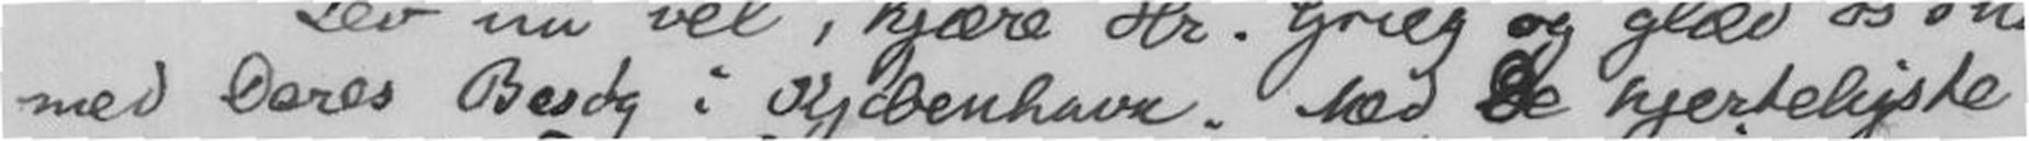med Deres Besøg i Kjøbenhavn. De hjerteligste
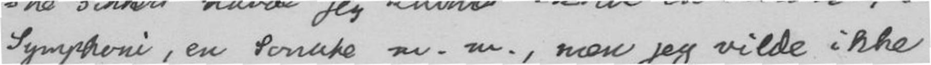Symphoni, en Sonate m.m., men jeg vilde ikke
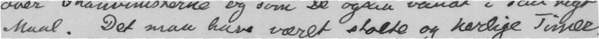Maal. Det maa have været stolte og herlige Timer.
